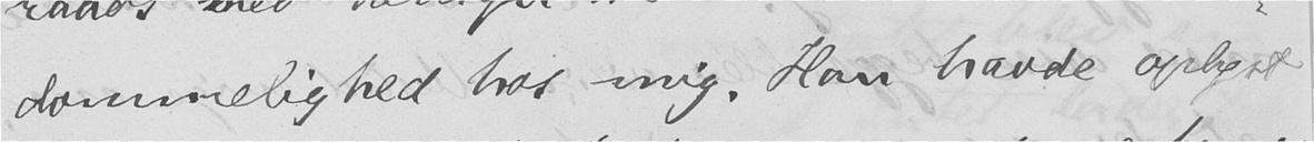dommelighed hos mig. Han havde oplyst
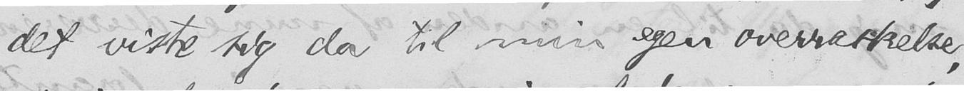det viste sig da til min egen overraskelse,
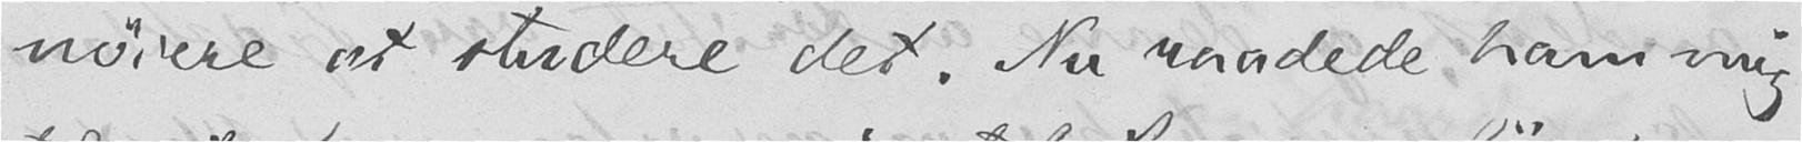nøiere at studere det. Nu raadede han mig
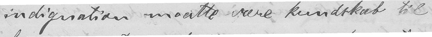indignation maatte være kundskab til
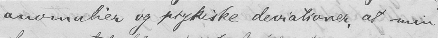anomalier og psykiske deviationer, at min
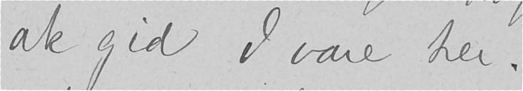ak gid I vare her.
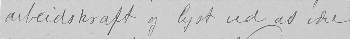arbeidskraft og lyst ved at være
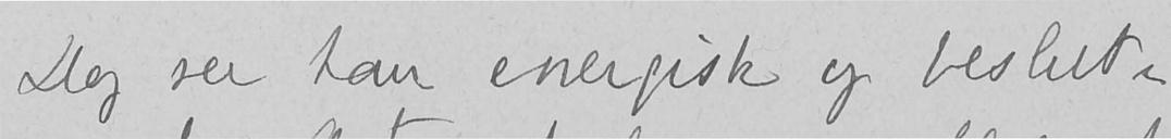Dog ser han energisk og beslut-
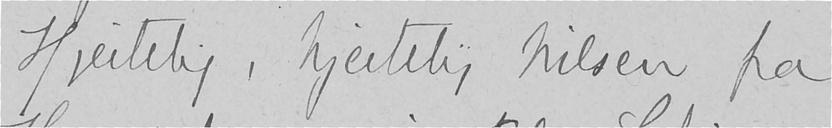Hjertelig, hjertelig hilsen fra
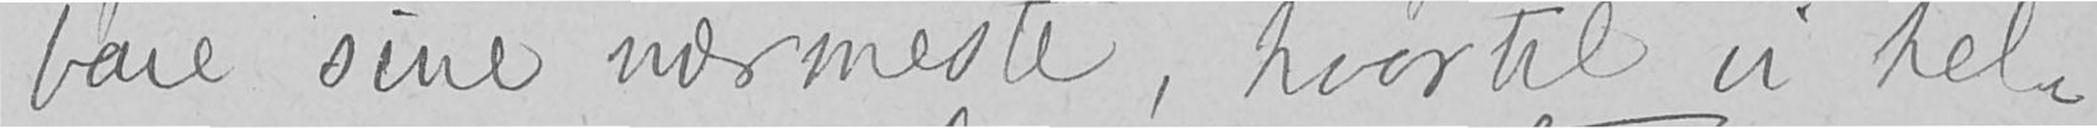bare sine nærmeste, hvortil vi hel-
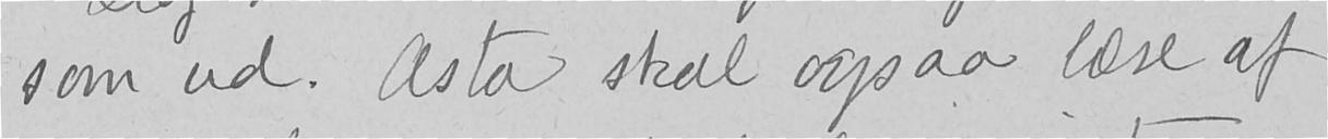som ud. Asta skal oogsaa læse af
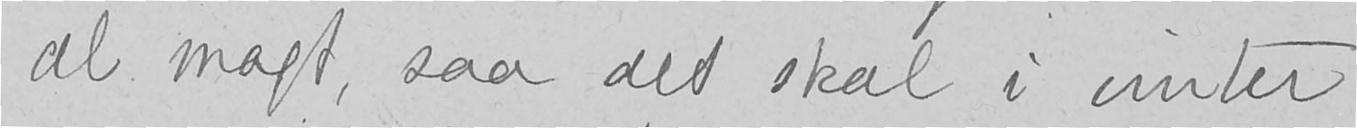al magt, saa det skal i vinter
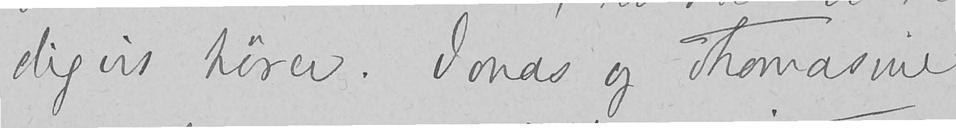digvis hører. Jonas og Thomassn,
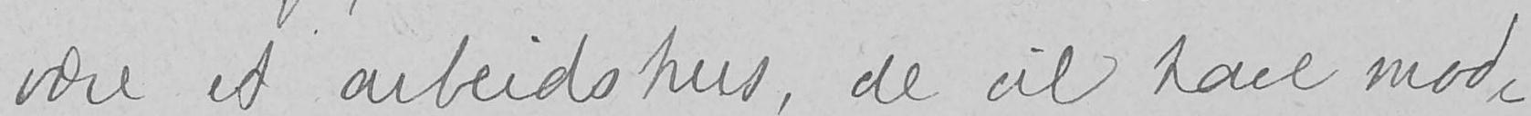være et arbeidshus, de vil have mod-
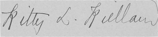Kitty L. Kielland
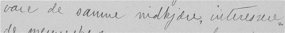vare de samme nidkjære, interessere-
